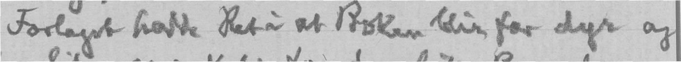Forlaget hadde Ret i at Boken blir for dyr og
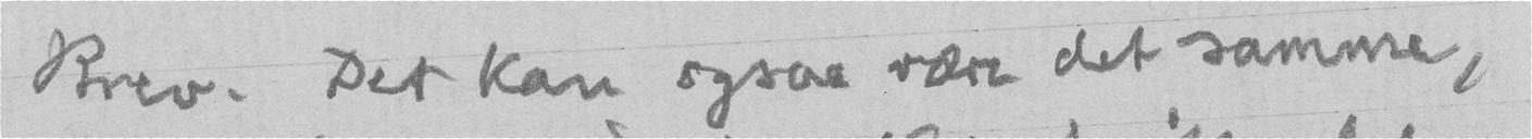Brev. Det kan ogsaa være det samme,
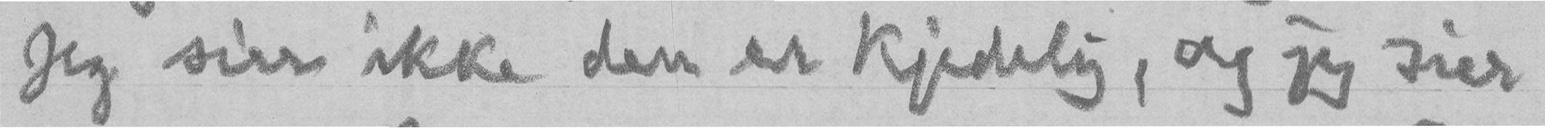Jeg sier ikke den er kjedelig, og jeg sier
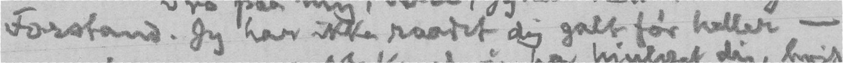Forstand. Jeg har ikke raadet dig galt før heller -
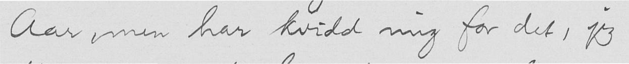Aar men har kvidd mig for det, jeg
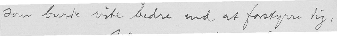som burde vite bedre end at forstyrre dig,
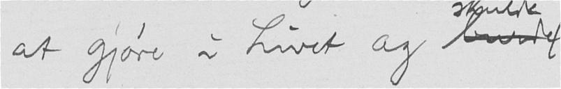at gjøre i Livet og burde
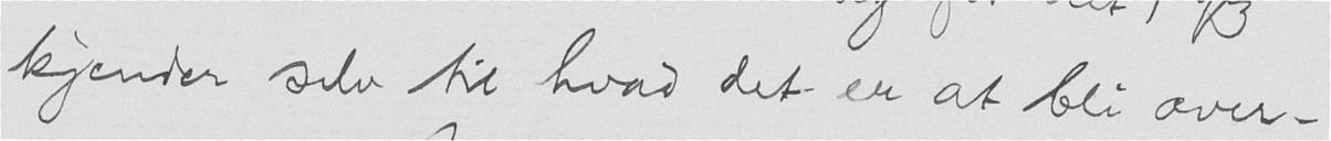kjender selv til hvad det er at bli over-
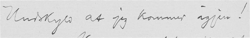Undskyld at jeg kommer igjen!
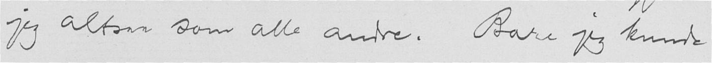jeg altsaa som alle andre. Bare jeg kunde
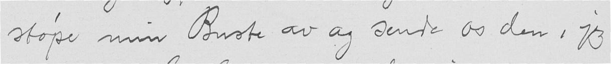støpe min Buste av og sende os den, jeg
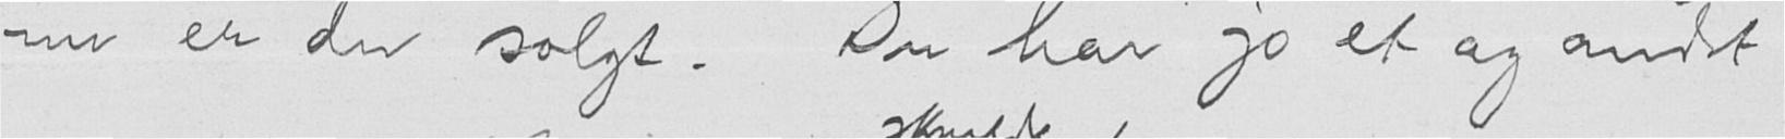nu er du solgt. Du har jo et og andet
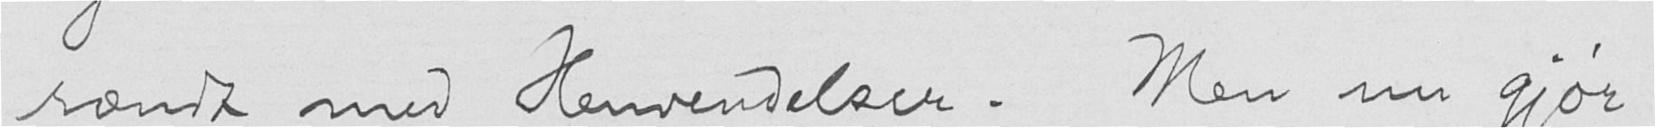rændt med Henvendelser. Men nu gjør
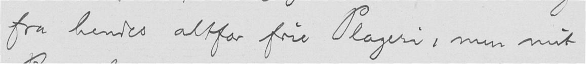fra hendes altfor frie Plageri, men mit
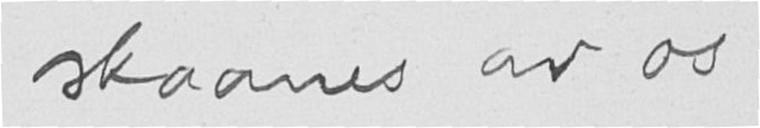skaanes av os
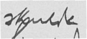skulde
